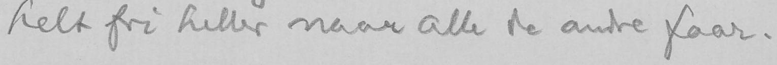helt fri heller naar alle de andre faar.
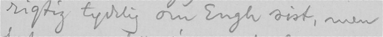rigtig tydelig om Engh sist, men
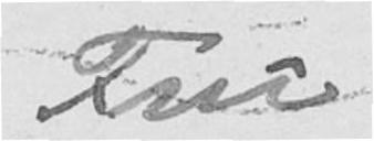Fru
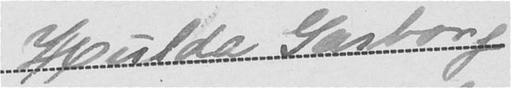Hulda Garborg
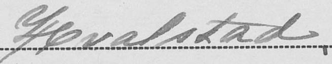Hvalstad
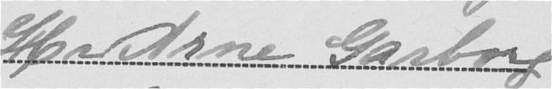Hr. Arne Garborg
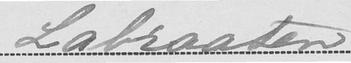Labraaten
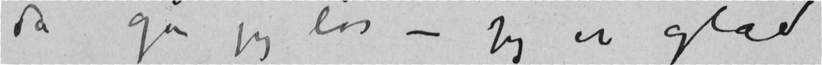da går jeg løs – Jeg er glad
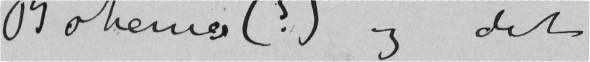Bohemer(?) og det
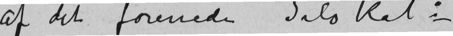af det forenede Selskab: –
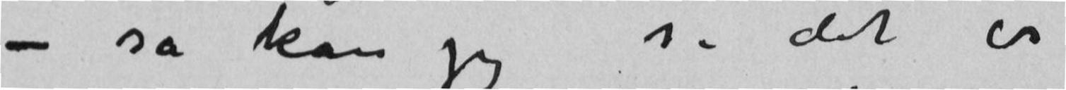– så kan jeg si det er
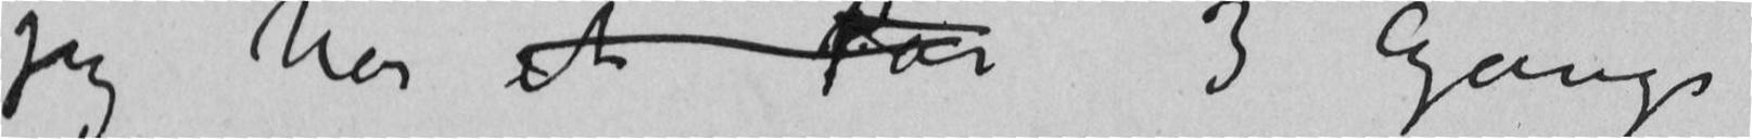jeg har et Par 3 Gange
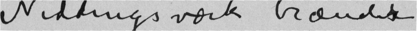Niddingsværk brænder
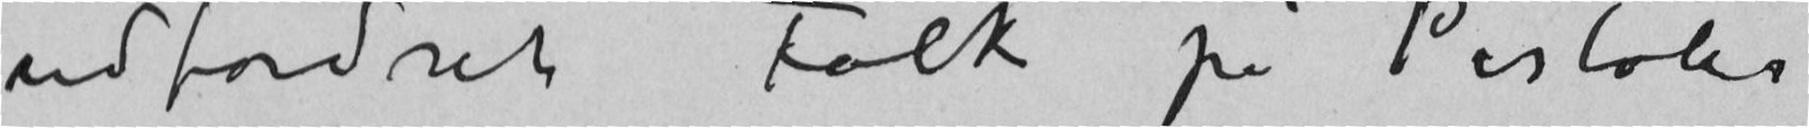udfordret Folk på Pistoler
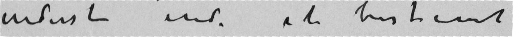inderst inde et bestemt
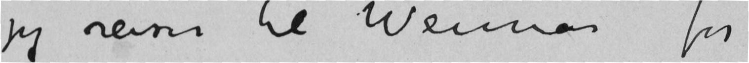jeg reiser til Weimar for
